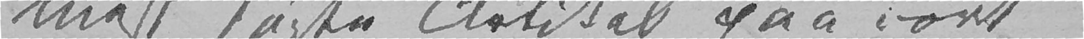mest søgte Artikel paa vort
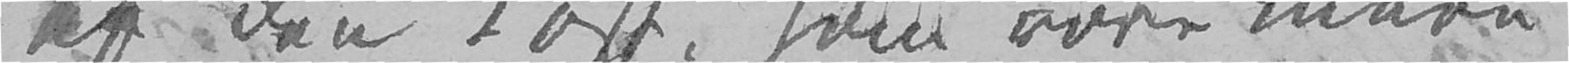af den Kost, som vore mere
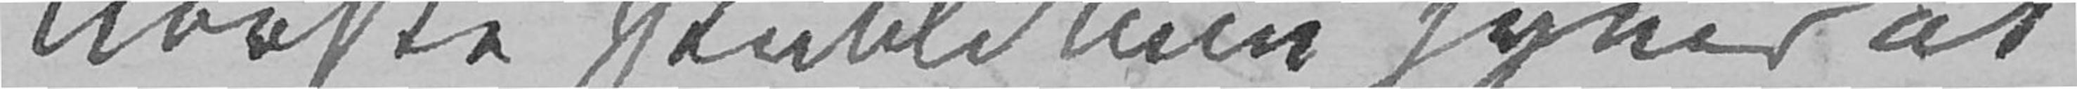norske Publikum synes at
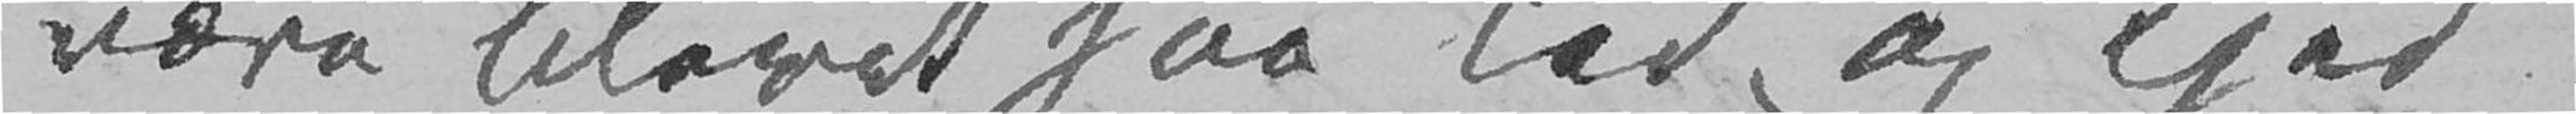være blevet saa led og kjed
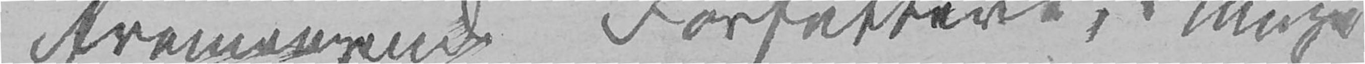fremragende Forfattere, i lange
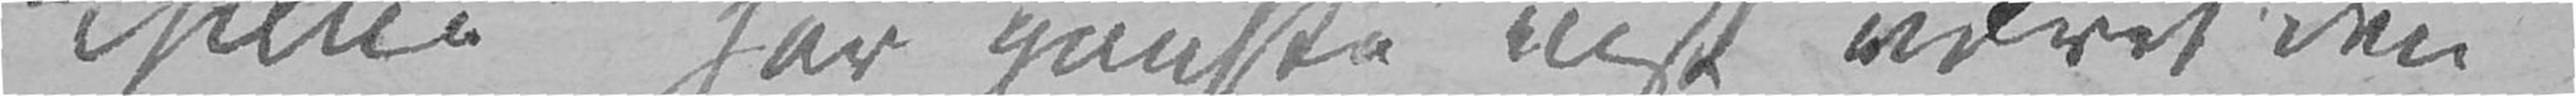Gillie» har ganske vist været den
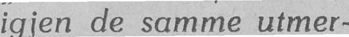igjen de samme utmer-
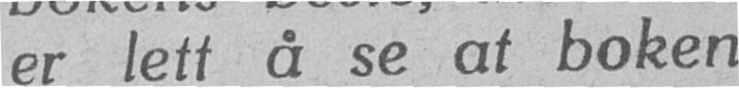er lett se at boken
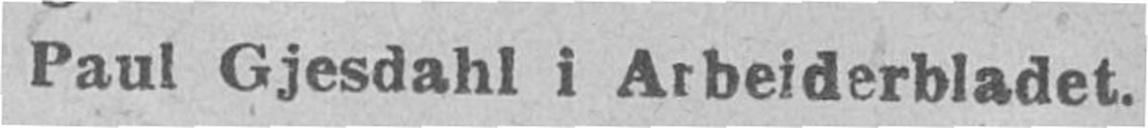Paul Gjesdahl i Arbeiderbladet.
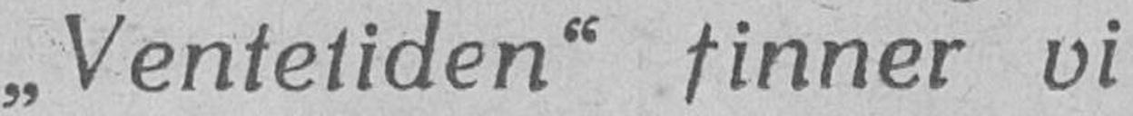"Ventetiden" finner vi
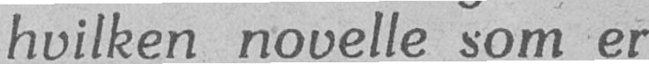hvilken novelle som er
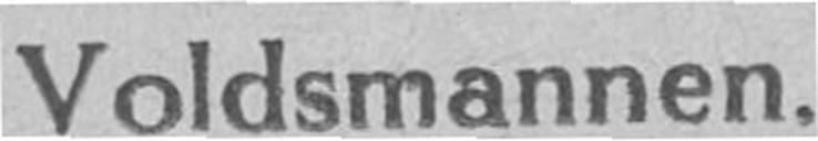Voldsmannen.
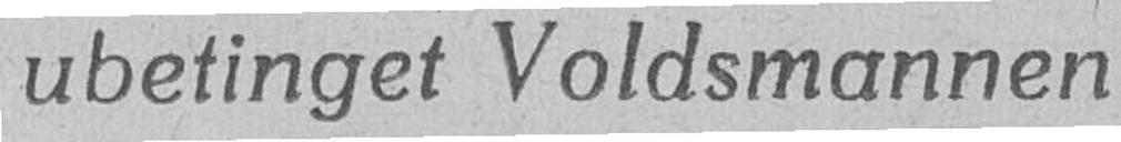ubetinget Voldsmannen
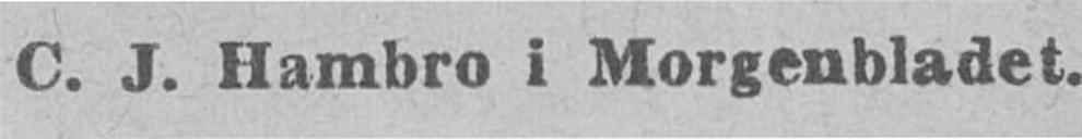C. J. Hambro i Morgenbladet.
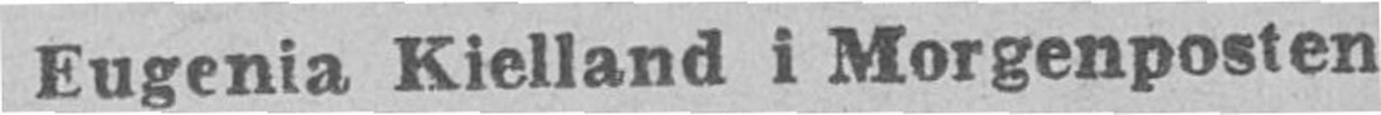Eugenia Kielland i Morgenposten
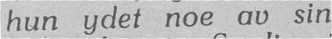hun ydet noe av sin
